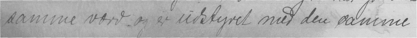samme værd og er udstyret med den samme
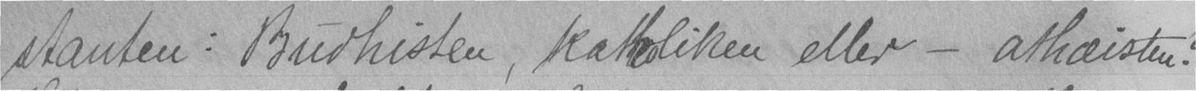stanten: Budhisten, katholiken eller - athæisten?
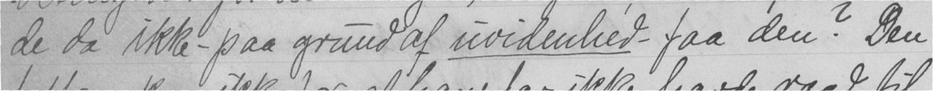de da ikke- paa grund af uvidenhed faa den? Den
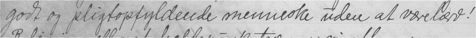godt og pligtopfyldende menneske uden at være lærd!
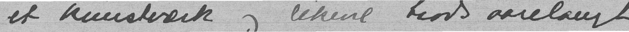et kunstværk og likevel trods aarelangt
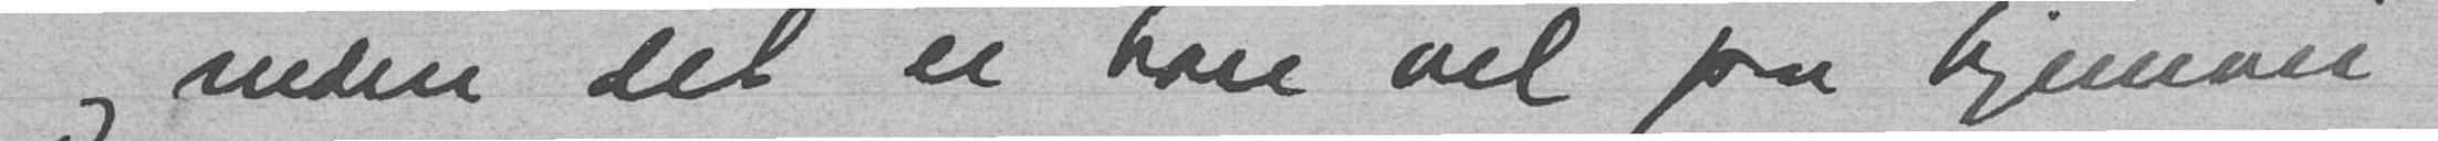og inden det er han vel paa hjemvei.
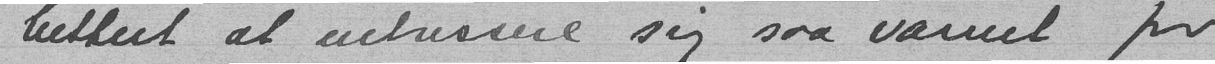bittrest at interesserer sig saa varmt for
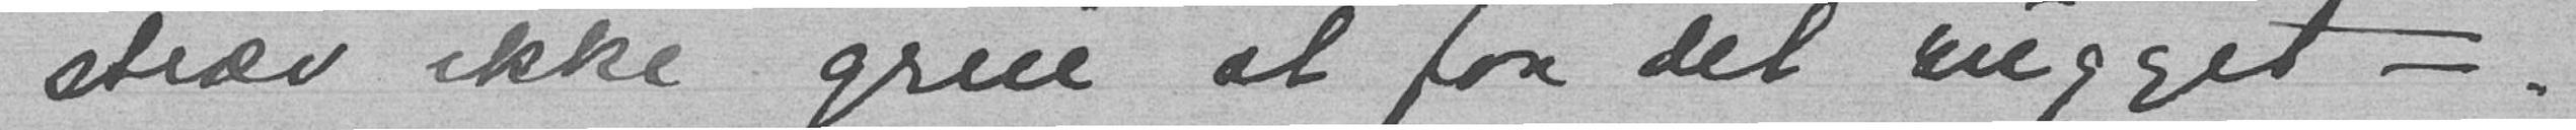stræv ikke greie at faa det hugget -.
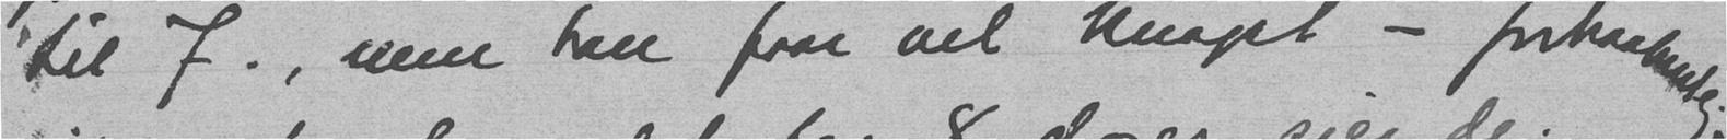til J., men han faar vel knapt - forhaabentlig
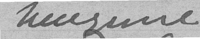hengivne
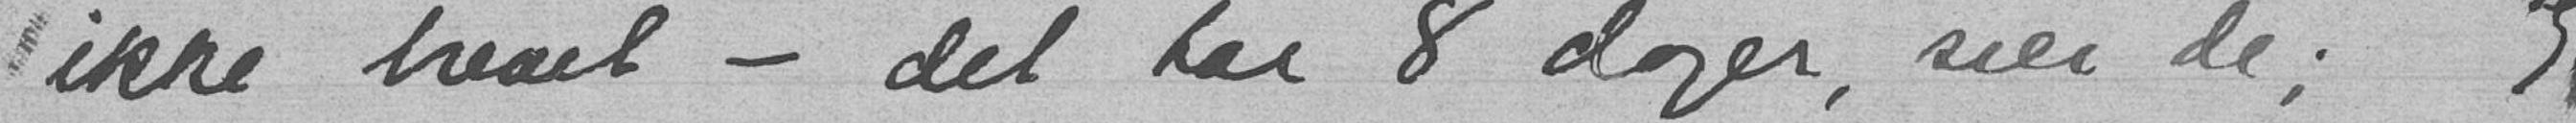ikke brevet - det tar 8 dage, sier de;
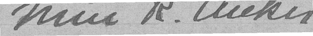Nini R. Anker
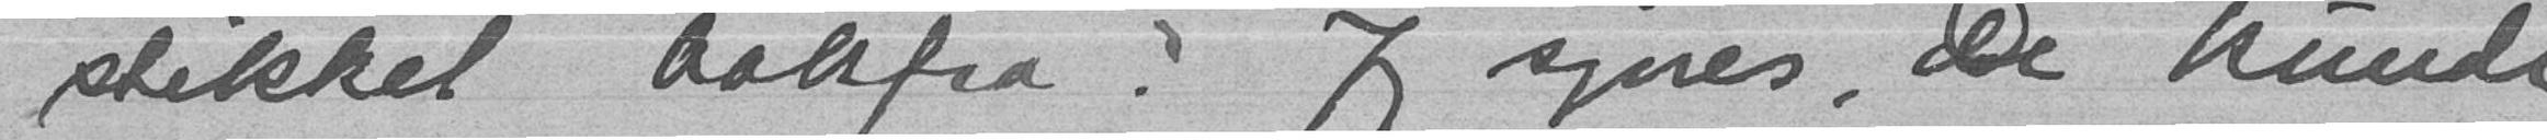stikket bakfra? Jeg synes, De kunde
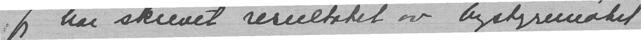Jeg har skrevet resultatet av bystyremøtet
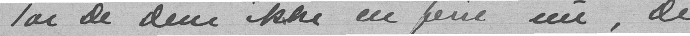Tar De Dem ikke en ferie nu, De
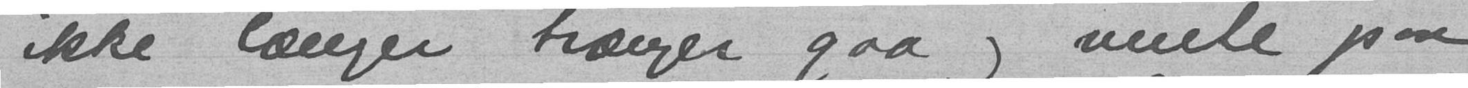ikke længer trænger gaa og vente paa
